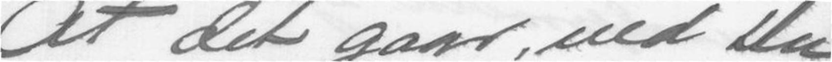At det gaar, ved Du
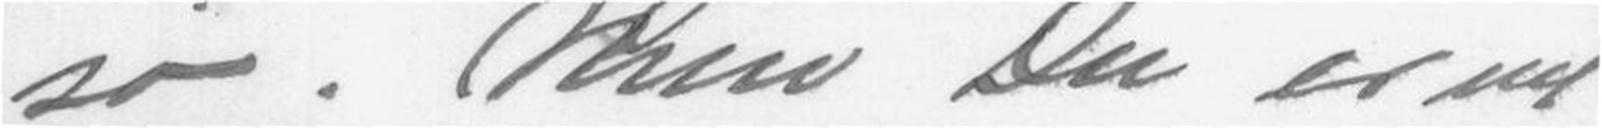jo. Men Du er vel
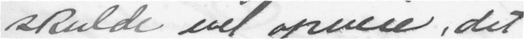skulde vel opveie, det
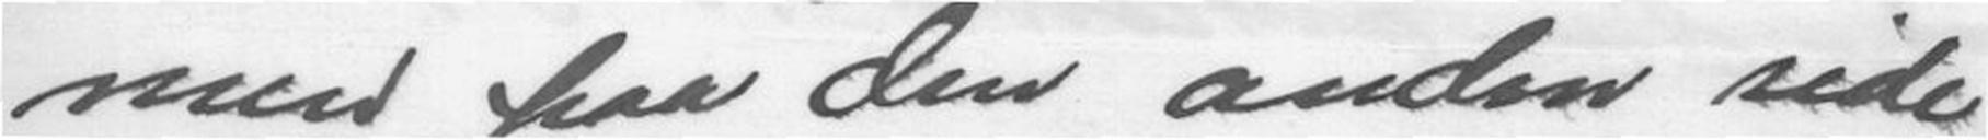men paa den anden side
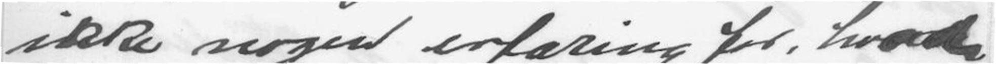ikke nogen erfaring for, hvordan
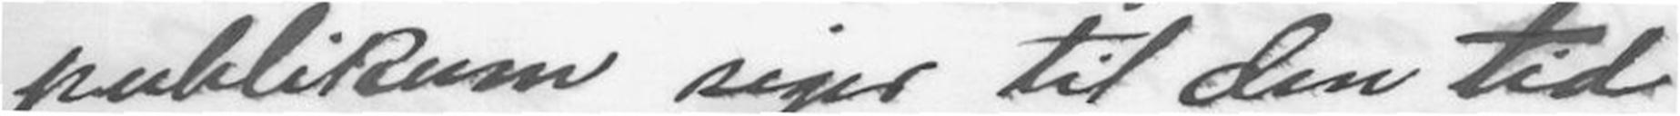publikum siger til den tid
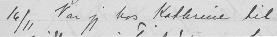16/11 Var jeg hos Kathrine til
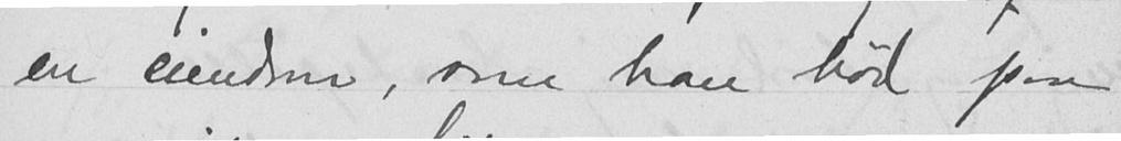en eiendom, som han bød paa
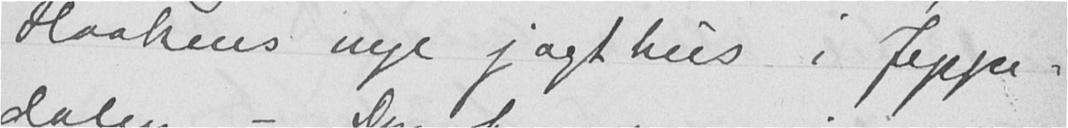Haakens nye jagthus i Jeppe-
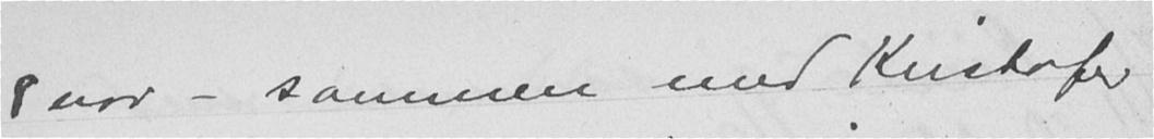8 nov - sammen med Kristofer
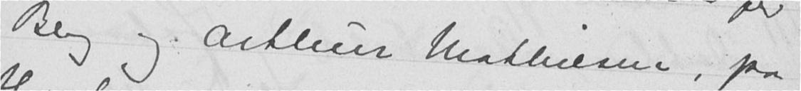Berg og Arthur Mathiesen, paa
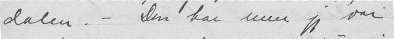dalen. - Don har men jeg var
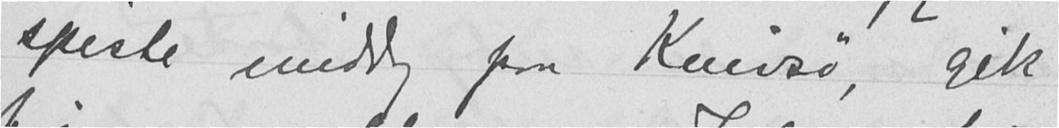spiste middag paa Knivsø, gik
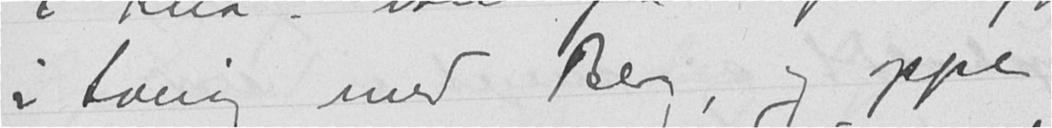i Sverig med Berg, og oppe
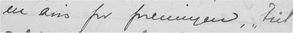en avis for foreningen, "Frit
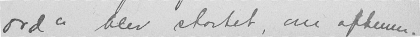ord" blev startet, om aftenen.
